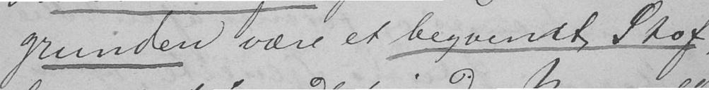grunden være et beqvemt Stof,
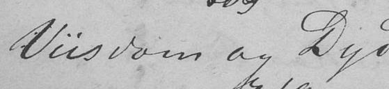Viisdom og Dyd
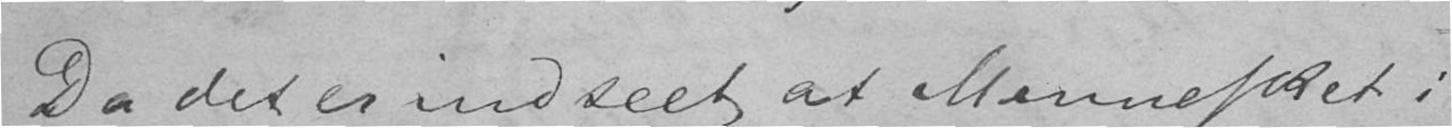Da det er indseet, at Mennesket i
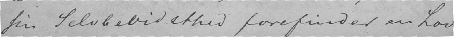sin Selvbevidsthed forefinder en Lov
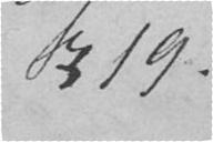§ 19.
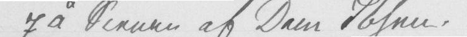på Scenen af Dem Ibsen.
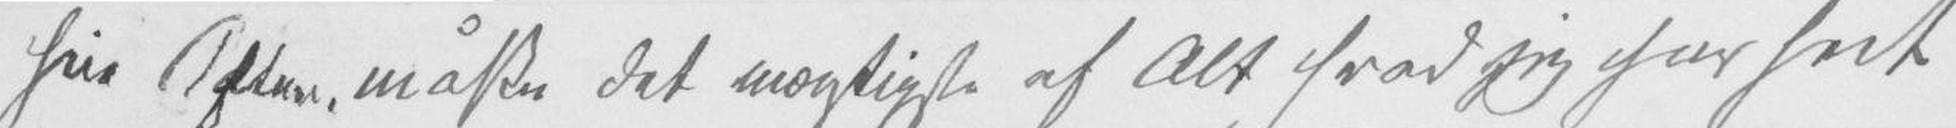hin Aften, måske det mægtigste af Alt hvad jeg har seet
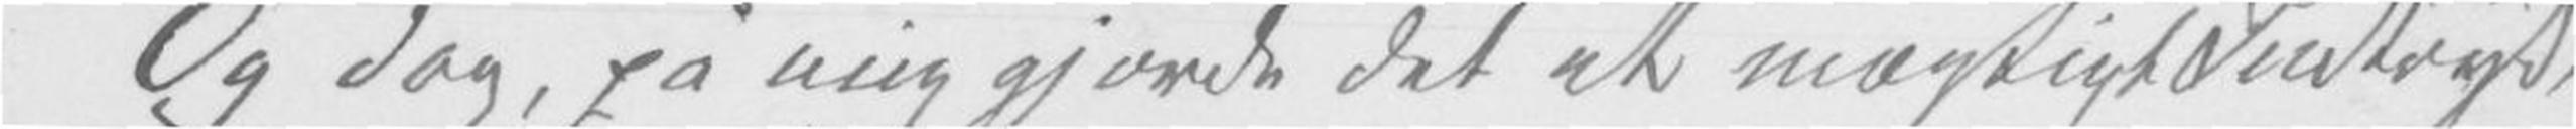Og dog, på mig gjorde det et mægtigt Indtryk,
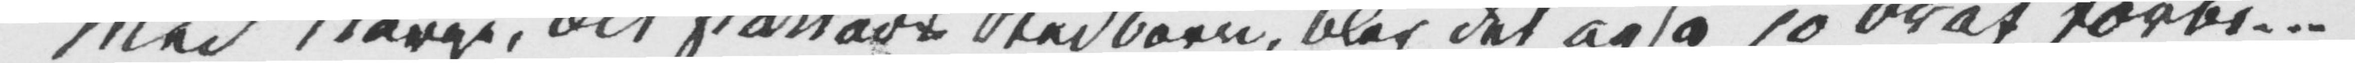Med Norge, dit stakkers Stedbarn, blev det også jo brat forbi ...
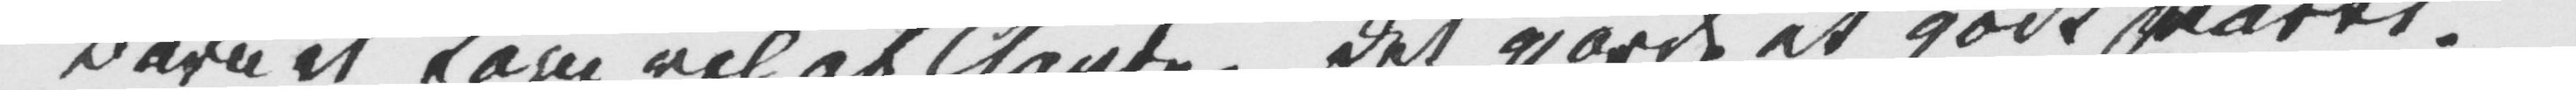Barnet kom vel af Gårde. Det gjorde et godt Parti.
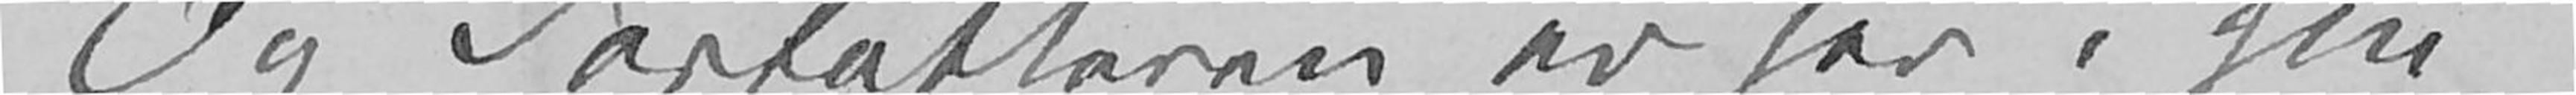Og Forfatteren er her i sin
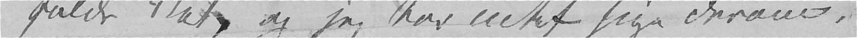fulde Ret, og jeg tør intet sige derom.
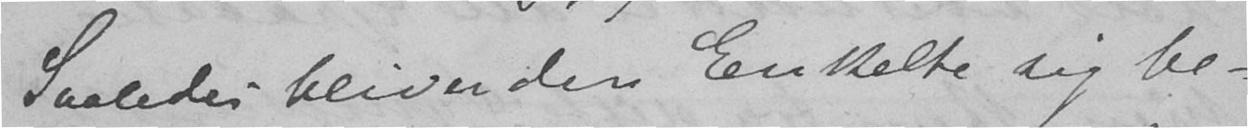Saaledes bliver den Enkelte sig be-
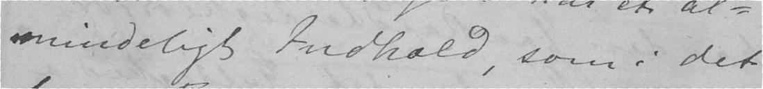mindeligt Indhold, som i det
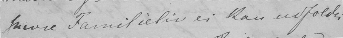hiere Familieliv ei kan udfoldes.
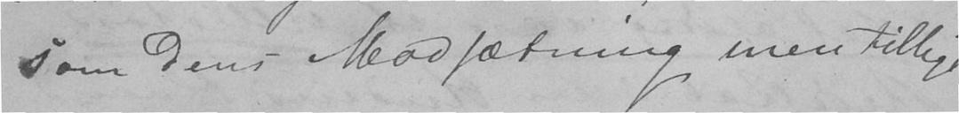som dens Modsætning, men tillige
